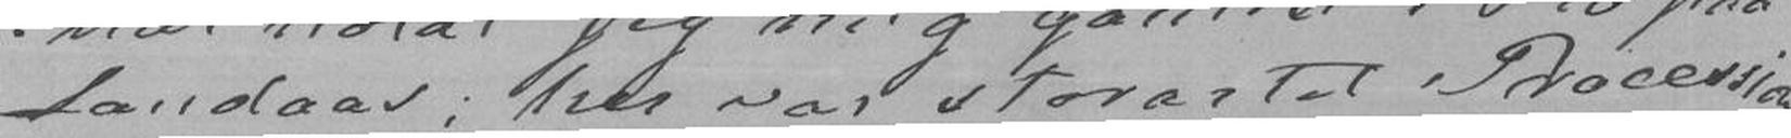Landaas; her var storartet Procession
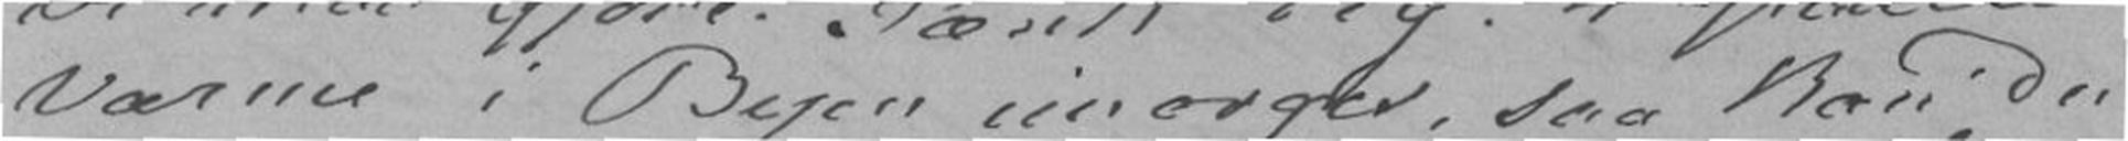Varme i Byen imorgen, saa kan Du
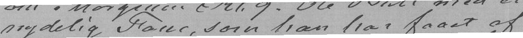nydelig Fane, som han har faaet af
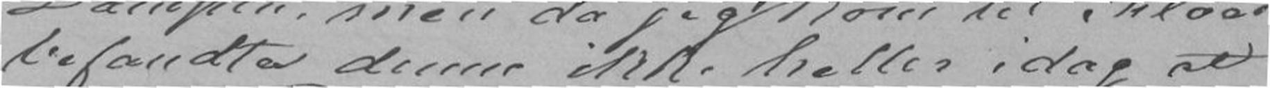befante denne ikke heller idag at
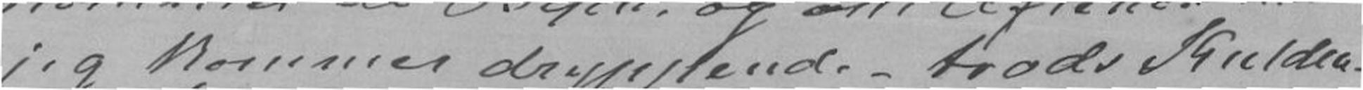jeg kommer dryppende - trods Kulden
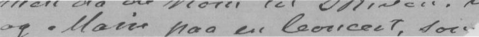og Marie paa en Concert, so
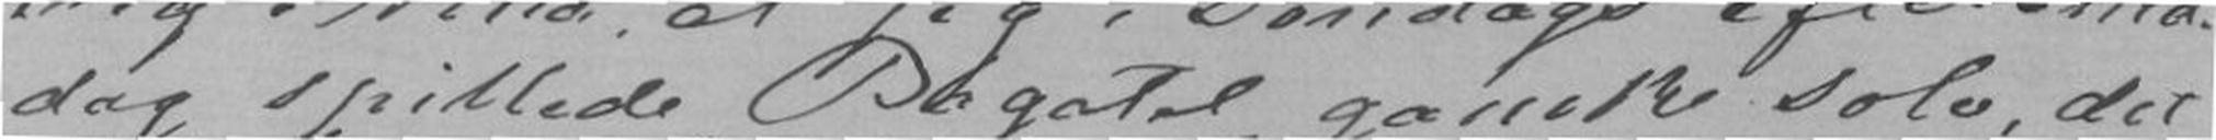dag spillede Bagatel ganske selv, det
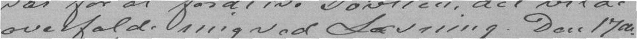overfalde mig ved Læsning. Den 17de
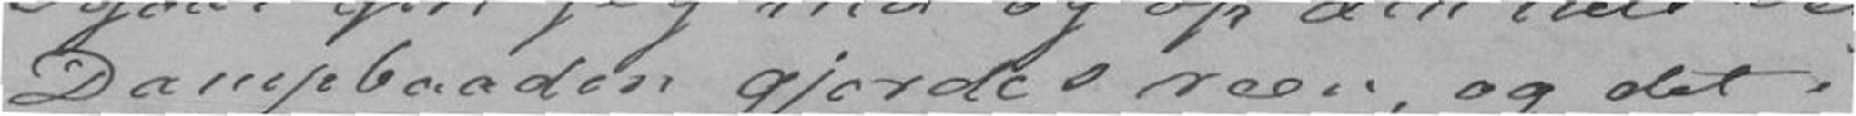Dampbaaden gjorde reen, og det i
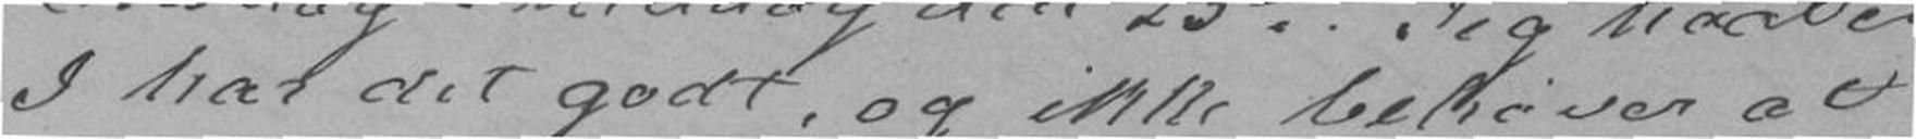I har det godt, og ikke behøver at
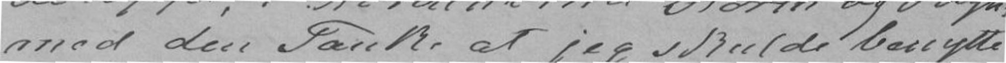med den Tanke at jeg skulde benytte
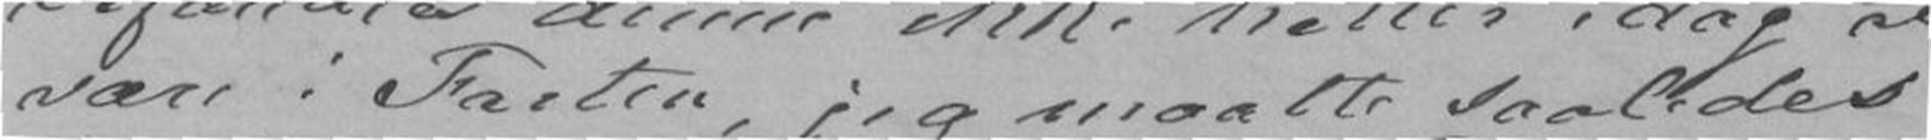være i Farten, jeg maatte saaledes
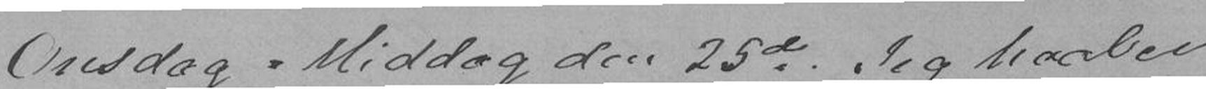Onsdag Middag den 25d. Jeg haaber
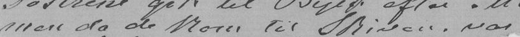men da de kom til Skiven var
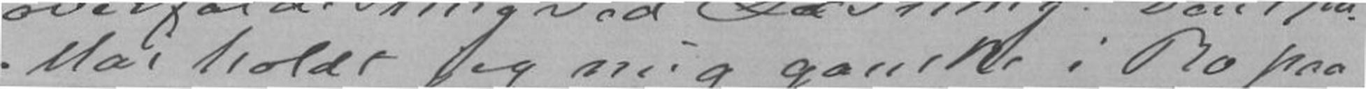Mai holdt jeg mig ganske i Ro paa
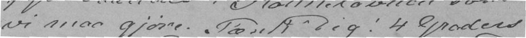vi maa gjøre. Tænk Dig! 4 Graders
